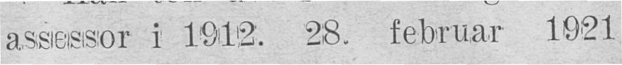assessor i 1912. 28. februar 1921
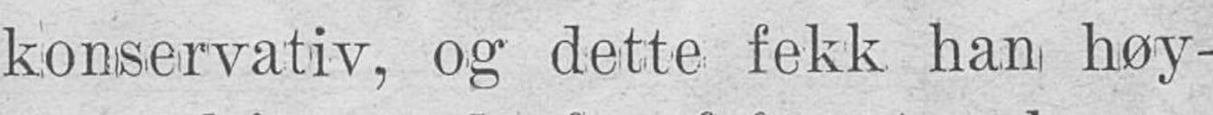konservativ, og dette fekk han høy-
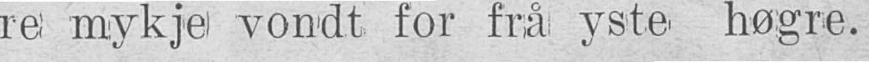re mykje vondt frå yste høgre.
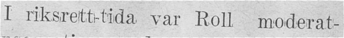I riksrett-tida var Roll moderat-
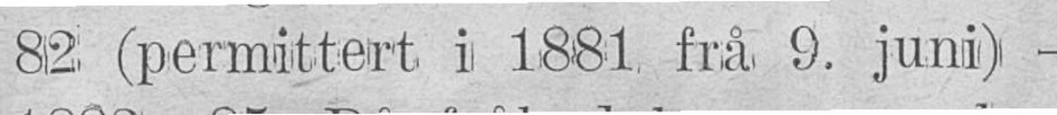82 (permittert i 1881 frå 9. juni) -
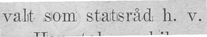valt som statsråd h. v.
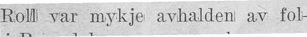Roll var mykje avhalden av fol-
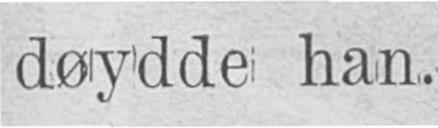døydde han.
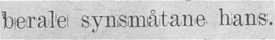berale synsmåtane hans.
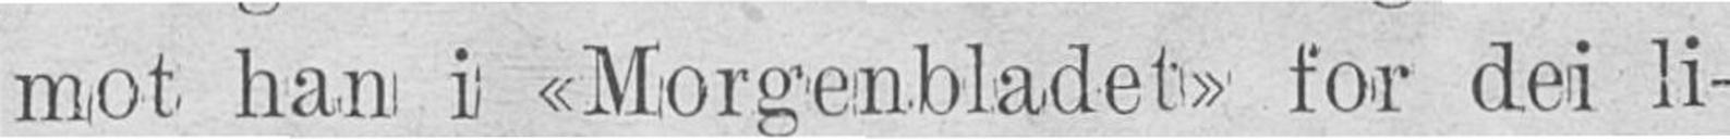mot han i "Morgenbladet" for dei li-
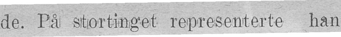de. På stortinget representerte han
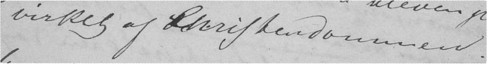virket af Christendommen.
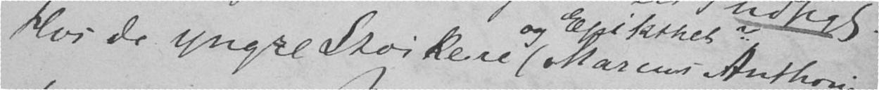Hos de yngre Stoikere (Marcus Aurelius
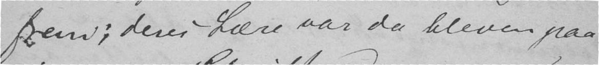frem; deres Lære var da bleven paa-
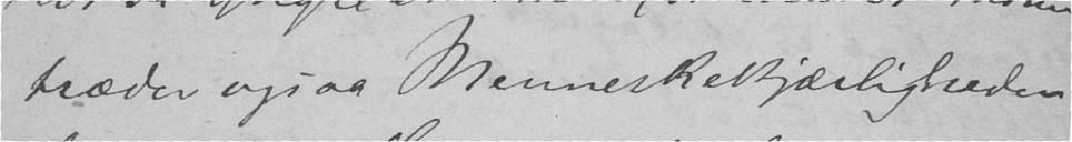træder ogsaa Menneskekjærligheden
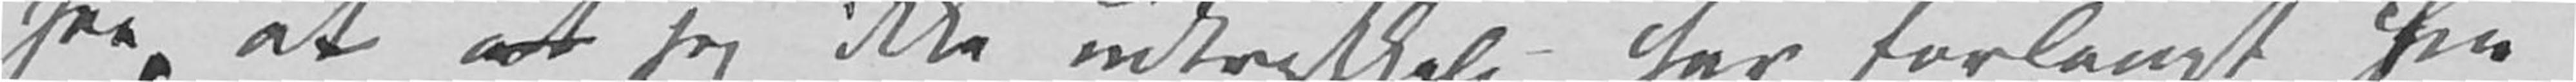see, at at jeg ikke udtrykkelig har forlangt hin
